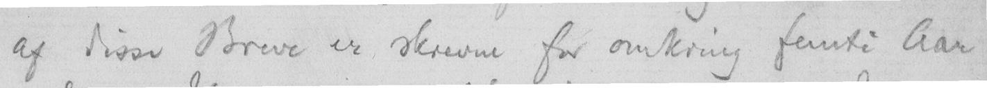af disse Breve er skrevne for omkring femti Aar
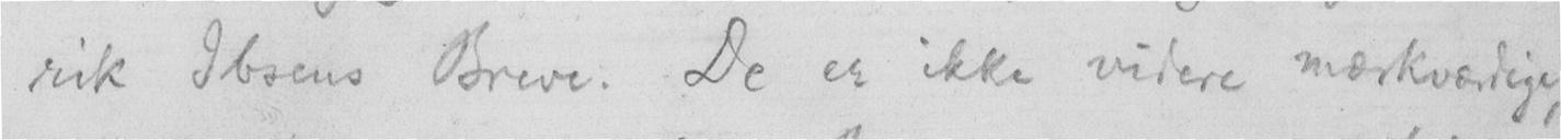rik Ibsens Breve. De er ikke videre mærkværdige,
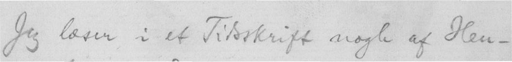Jeg læser i et Tidsskrift nogle af Hen-
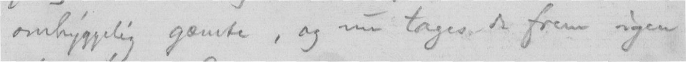omhyggelig gæmte, og nu tages de frem igen
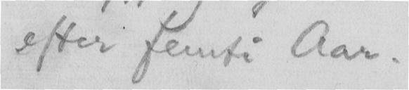efter femti Aar.
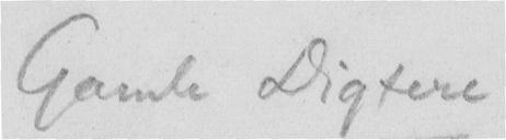Gamle Digtere
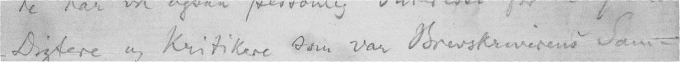Digtere og Kritikere som var Brevskriverens Sam-
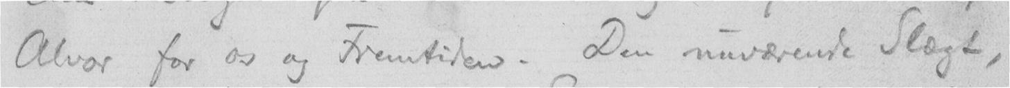Alvor for os og Fremtiden. Den nuværende Slægt,
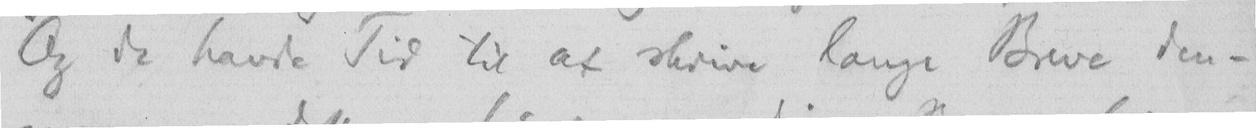Og de havde Tid til at skrive lange Breve den-
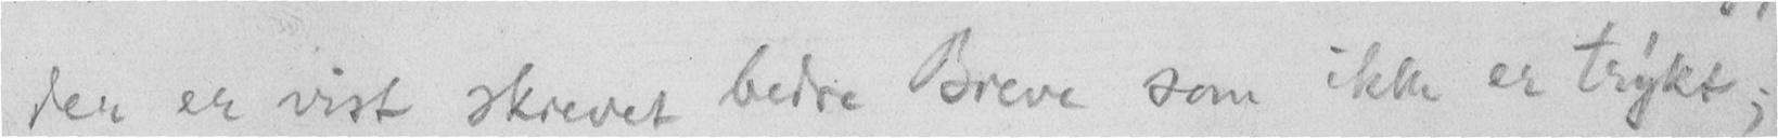der er vist skrevet bedre Breve som ikke er trykt;
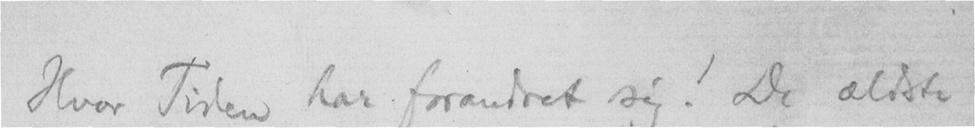Hvor Tiden har forandret sig! De ældste
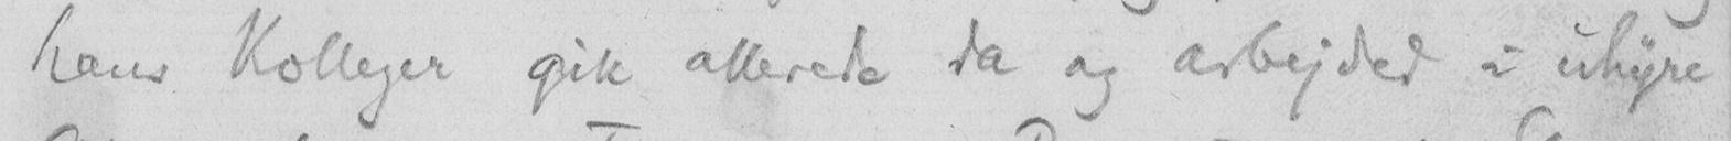hans Kolleger gik allerede da og arbejdet i uhyre
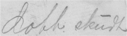Dobb ekudt
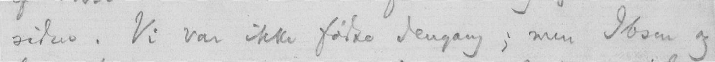siden. Vi var ikke fødte dengang; men Ibsen og
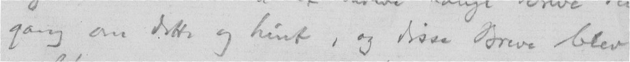gang om dette og hint, og disse Breve blev
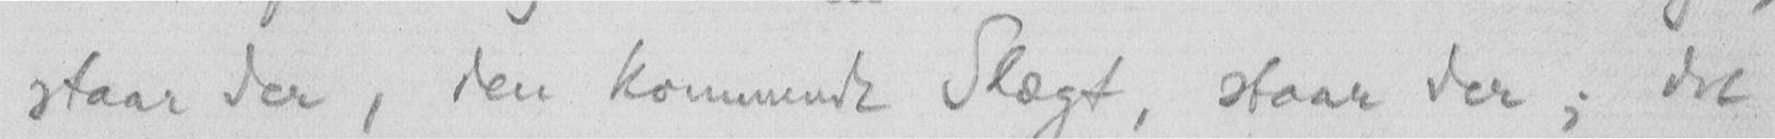staar der, den kommende Slægt, staar der; det
